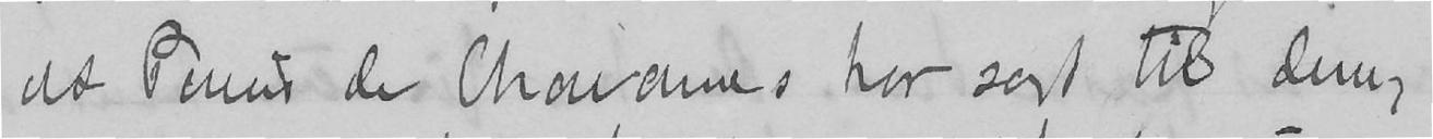at Puvis de Chavanne, har sagt til dem,
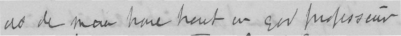at de maa have havt en god professeur
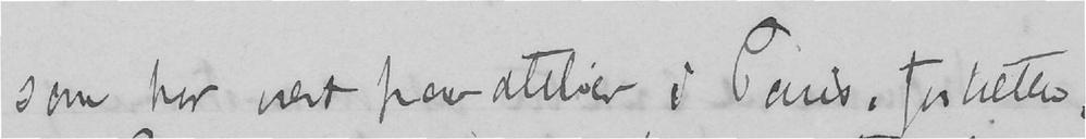som har vært paa atelier i Paris, fortæller,
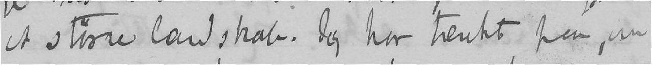et større landskab. Jeg har tænkt paa, om
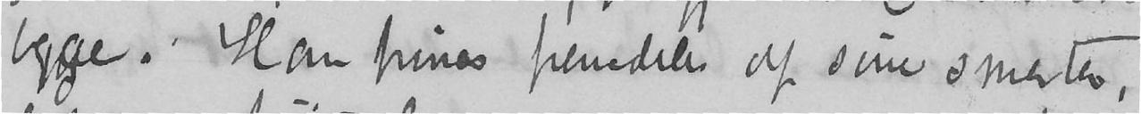begge. Han pines fremdeles af sine smerter,
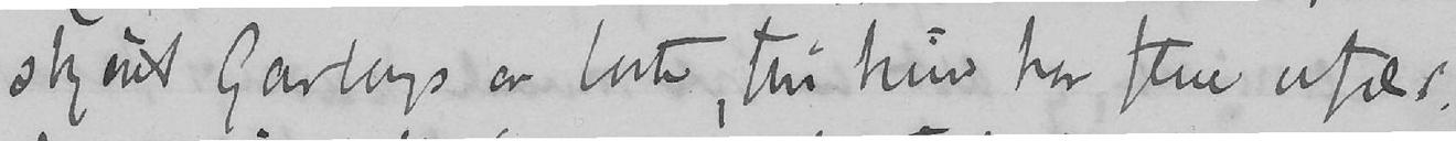skjønt Garborgs er borte, thi hun har flere ufær
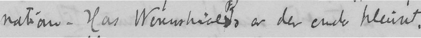nation. Hos Werenskiolds er der enda kleint.
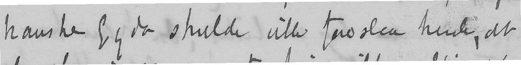kanske Gyda skulde ville foreslaa hende, at
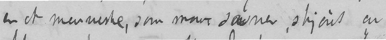er et menneske, som man savner, skjønt en
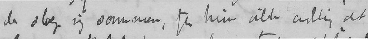de slog sig sammen, for hun vilde endelig, at
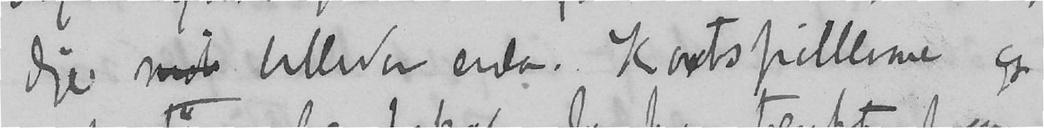dige moti billeder enda. Kortspillerne og
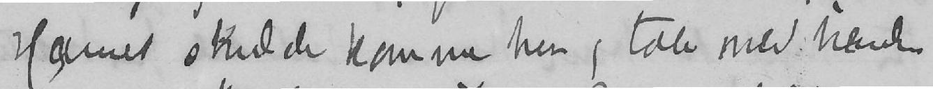Harriet skulde komme her og tale med hende
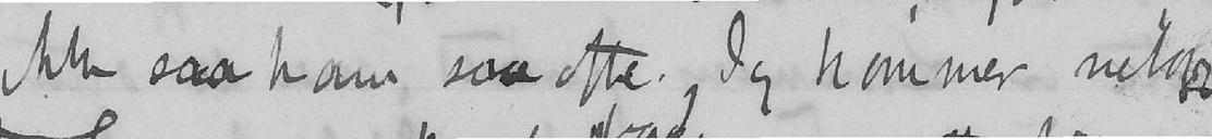ikke saa ham saa ofte. Jeg kommer
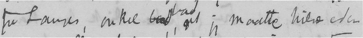netop fra Langes, onkel brad, at jeg maatte hilse eder
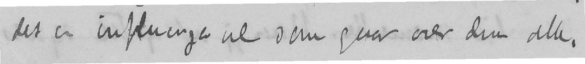Det er influenzen vel som gaar over dem alle.
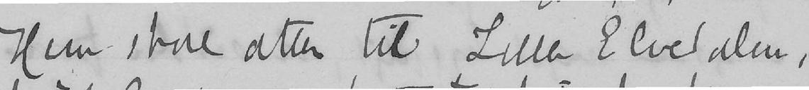Hun skal atter til Lille Elvedalen,
